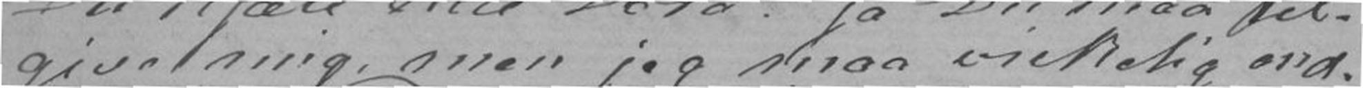give mig, men jeg maa virkelig end-
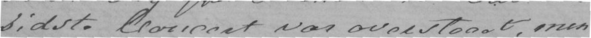sidste Concert var overstaaet, men
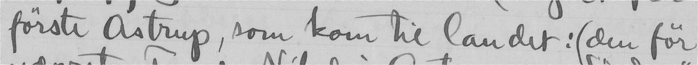forste Astrup, som kom til landet: (den før
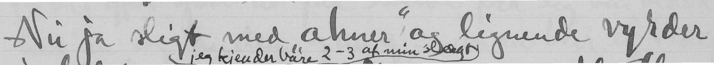Nu ja sligt med ahner og legnende vyrder
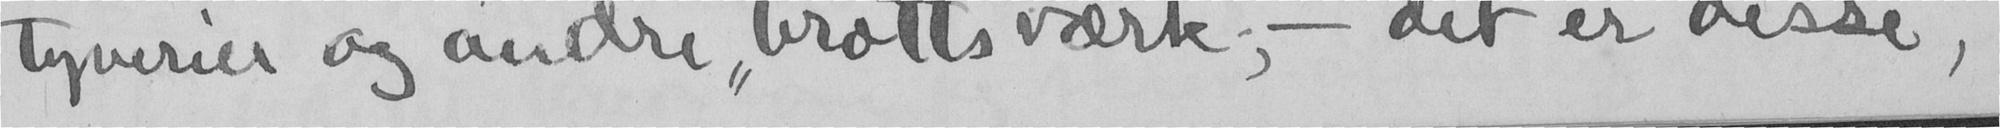tyverier og andre "brotts værk" - det er disse,
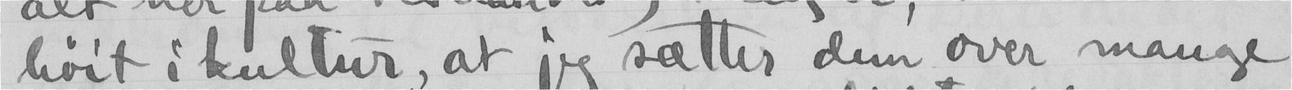høit i kultur, at jeg sætter dem over mange
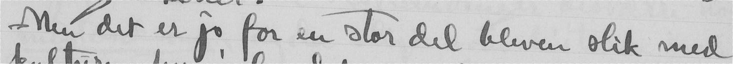Men det er jo for en stor del bleven slik med
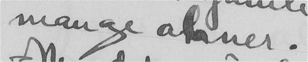mange ahner.
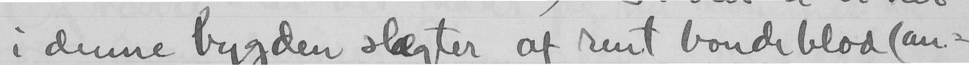i denne bygden slægter af rent bondeblod (an-
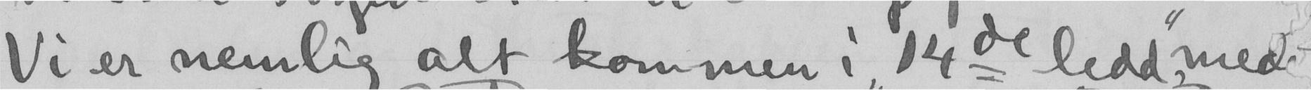Vi er nemlig alt kommen i 14de ledd medens
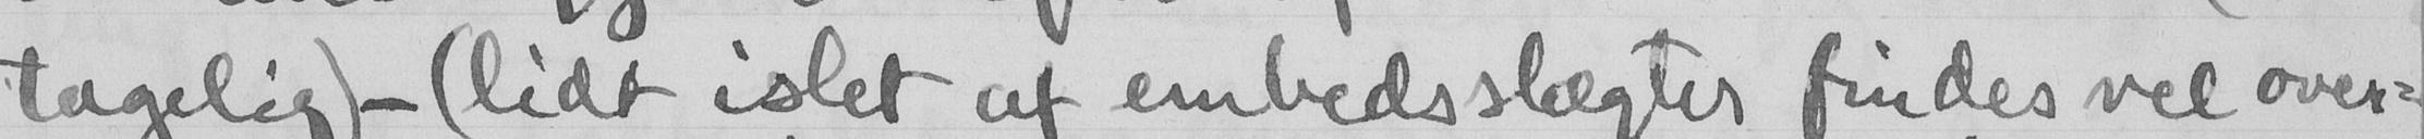tagelig) - (lidt islet af embedsslægter findes vel over-
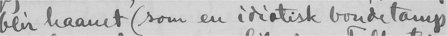blir haanet (som en idiotisk bonde tamp
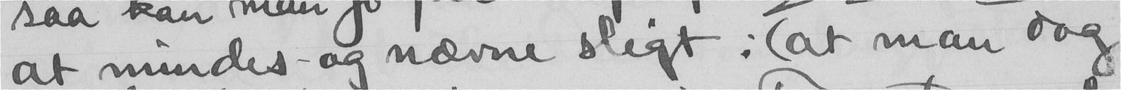at mindes - og nævne sligt: (at man dog
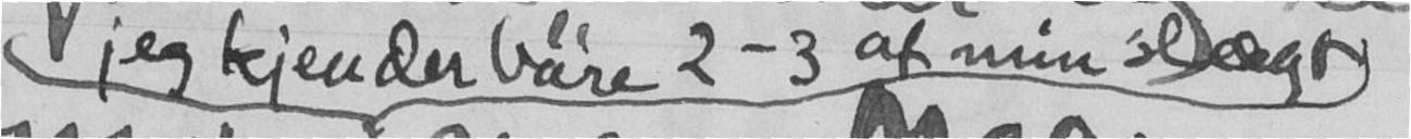jeg kjender bare 2-3 af min slægt
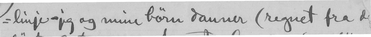- linje -jeg og mine børn danner (regnet fra di
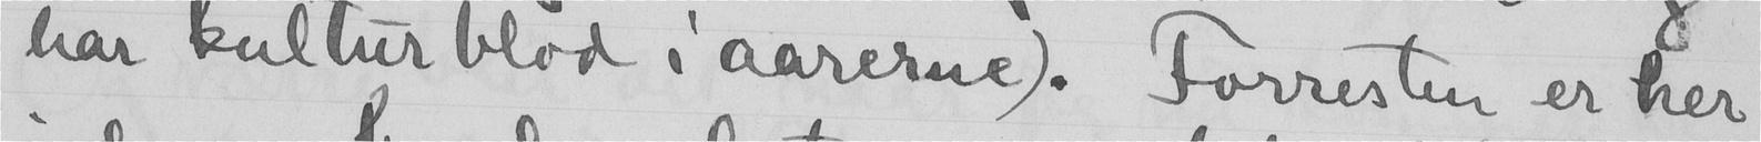har kulturblod i aarerne). Forresten er her
